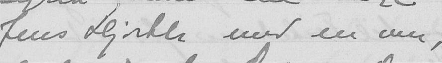Jens Hjorth med en ven,
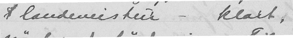l landeveistur - klart,
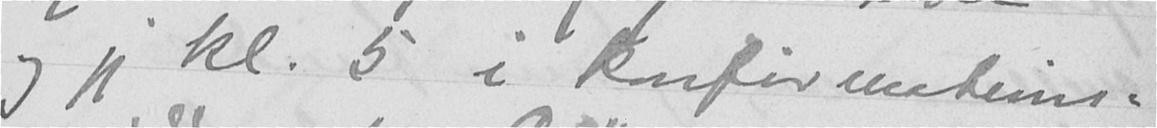og jeg kl. 5 i konfirmations-
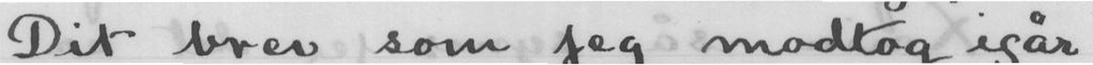Dit brev som jeg modtog igår
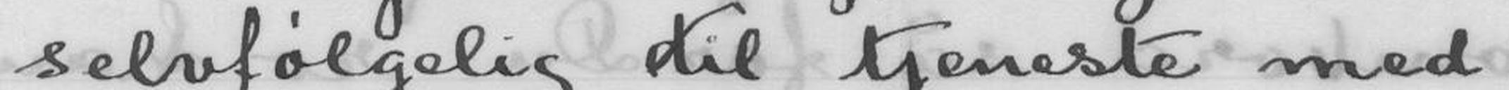selvfølgelig til tjeneste med
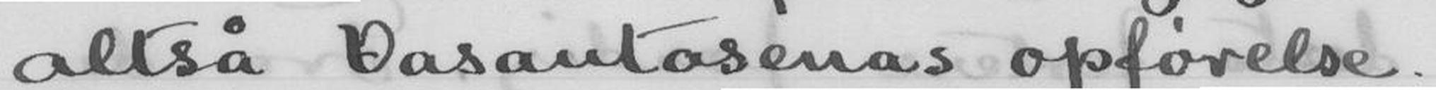altså Vasantasenas opførelse.
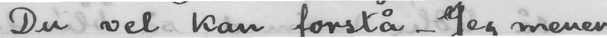Du vel kan forstå.- Jeg mener
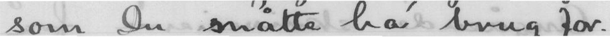som Du måtte ha' brug for.
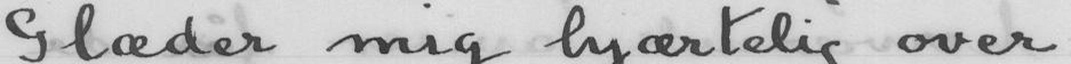Glæder mig hjærtelig over
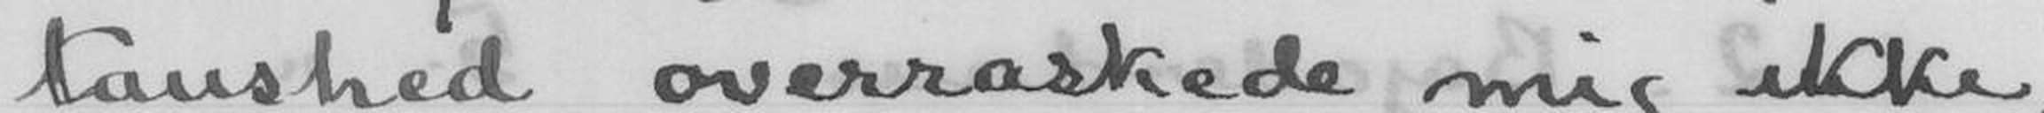taushed overraskede mig ikke,
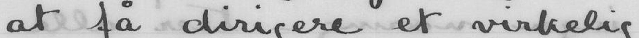at få dirigere et virkelig
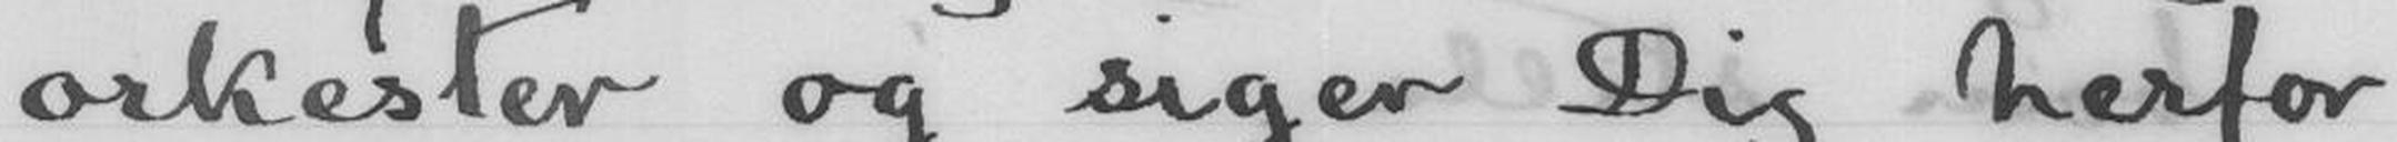orkester og siger Dig derfor
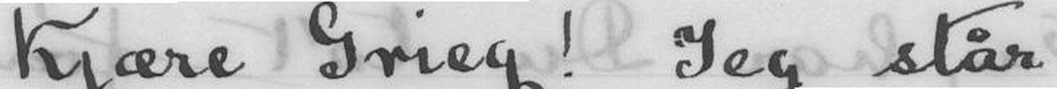Kjære Grieg! Jeg står
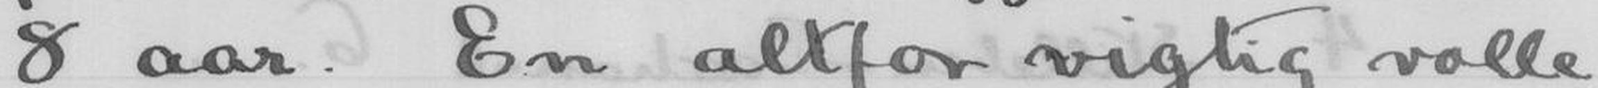8 aar. En altfor vigtig rolle
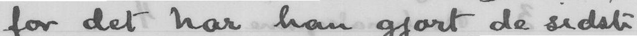for det har han gjort de sidste
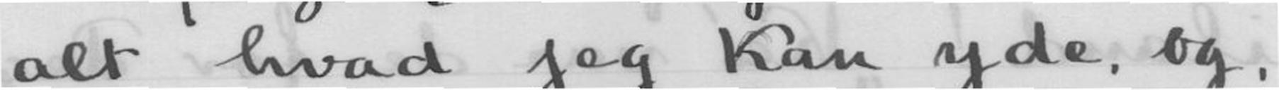alt hvad jeg kan yde, og,
